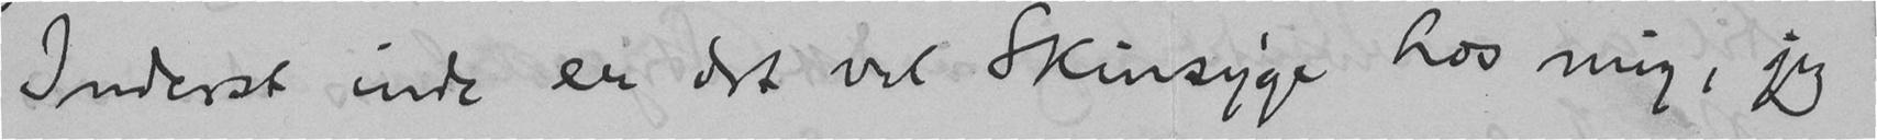Inderst inde er det vel Skinsyge hos mig, jeg
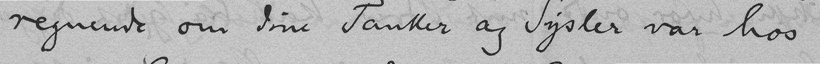regnende om dine Tanker og Sysler var hos
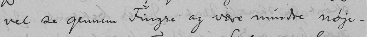vel se gennem Fingre og være mindre nøje-
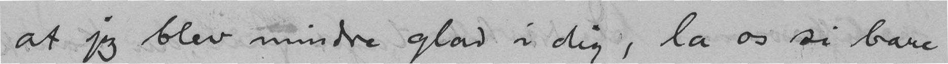at jeg blev mindre glad i dig, la os si bare
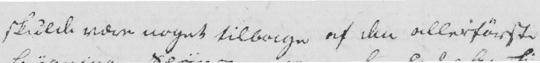skulde være noget tilbage af den allerførste
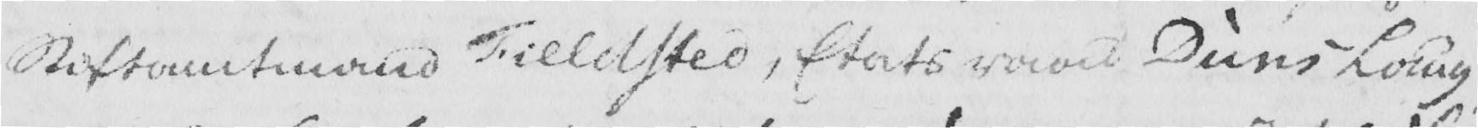Stiftamtmand Fieldsted, Etatsraad Duns Laug-
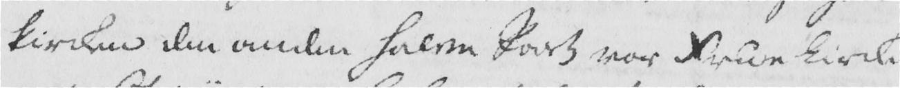kirken den anden halve Part vor Frue Kirke
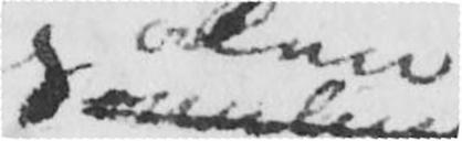Salen
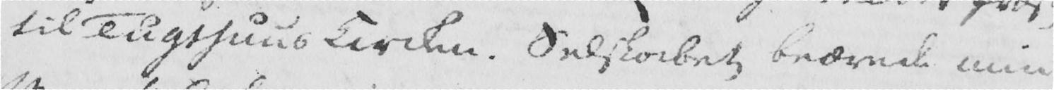til Tugthuus Kirken. Selskabet beærede min
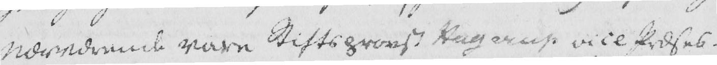Nærværende vare Stiftsprovst Hagerup vice Præses
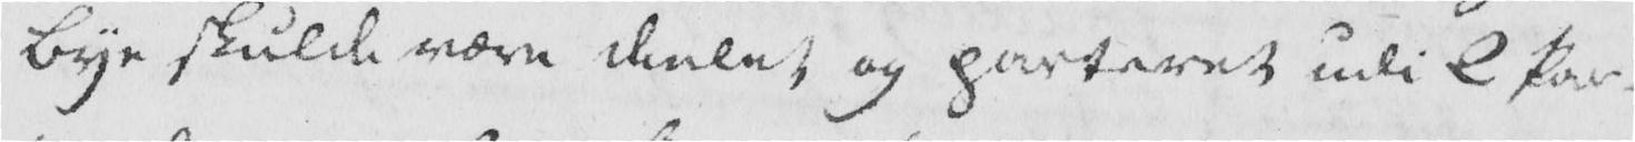Bye skulde være deelet og parteret udi 2 Par-
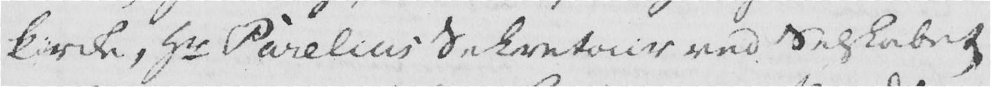kirke, Hr Parelius Sekretair ved Selskabet,
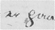er paa
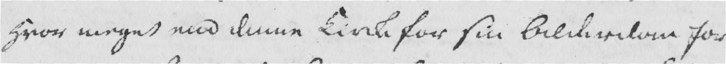Hvor meget end denne Kirke for sin Alderdom for-
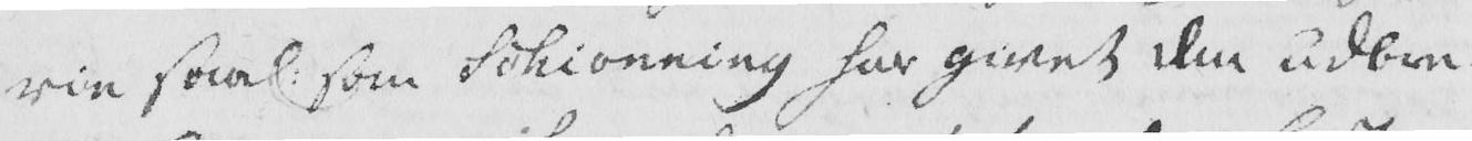rie saal. som Schiønning har givet den udbre-
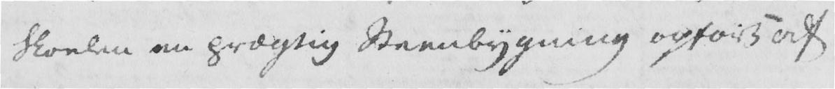Skolen en prægtig Steenbygning opført af
